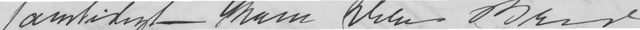samtidigt kom Deres Brev.
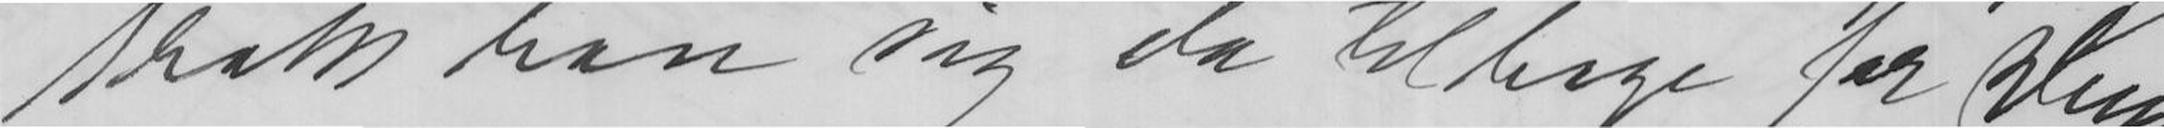trak han sig tilbage for Dem.
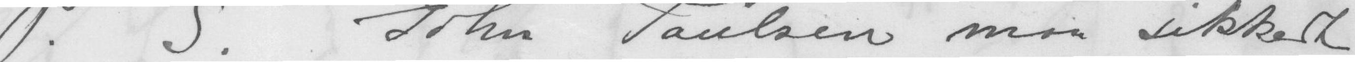P.S. John Paulsen maa sikkert
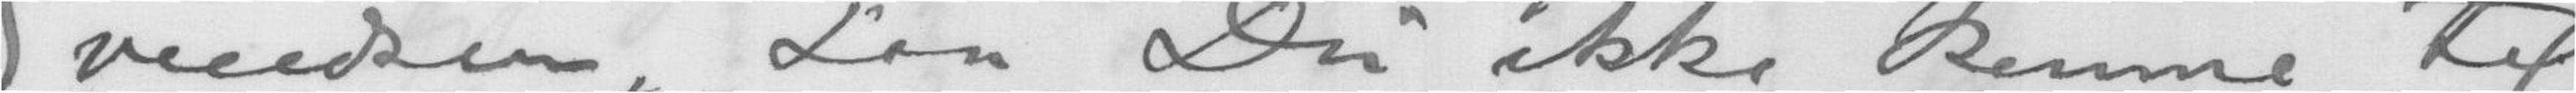Svendsen, saa Du ikke komme til
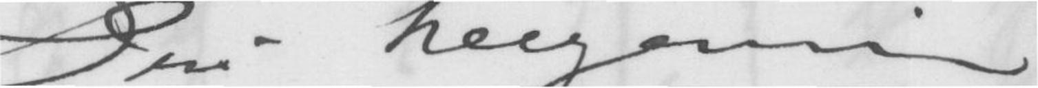Din hengivne
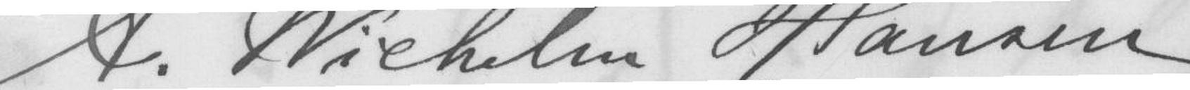A. Wilhelm Hansen
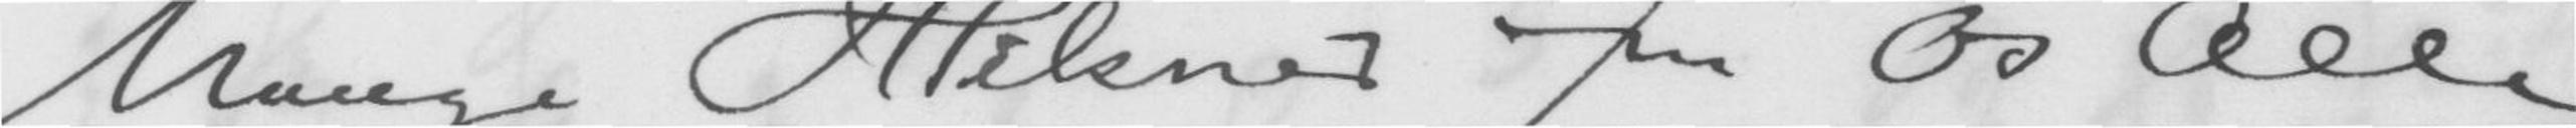Mange Hilsener fra os Alle
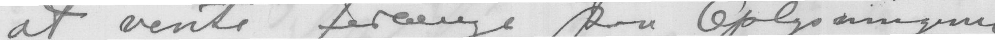at vente forlænge paa Oplysningene
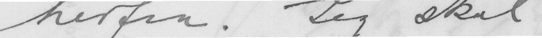herfra. Jeg skal
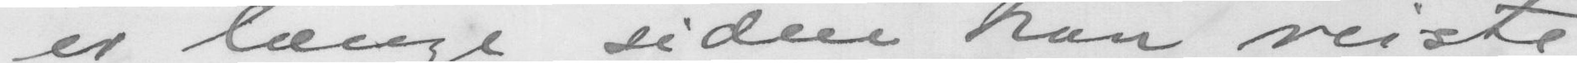er længe siden han reiste
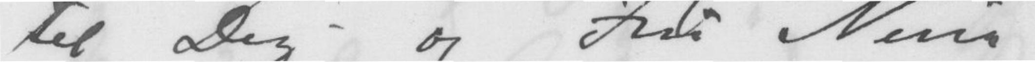til Dig og Fru Nina
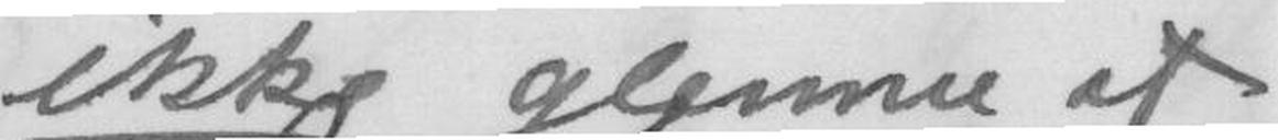ikke glemme at
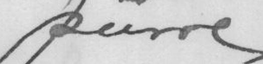purre
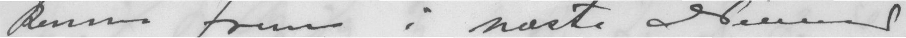komme frem i næste Maaned
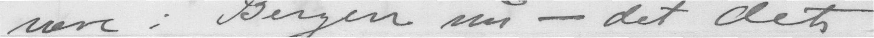være i Bergen nu - det
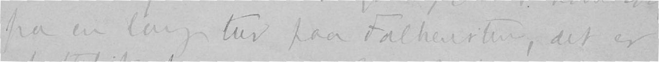fra en lang tur paa Falkensten, det er
